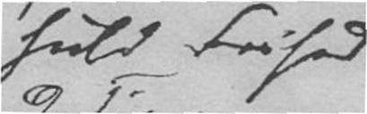fuld Frihed
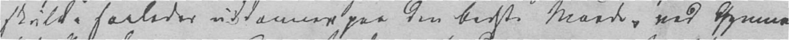skulde saaledes uddannes paa den bedste Maade, ved Gymna-
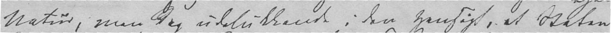Natur, men dog udelukkende i den Hensigt, at Staten
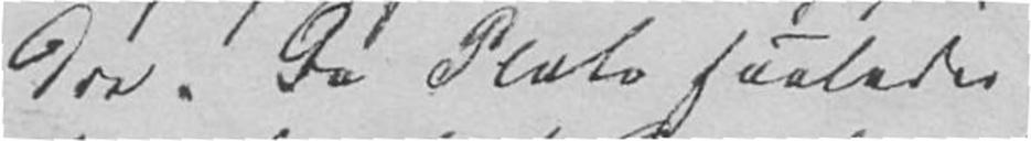dre. Da Plato saaledes
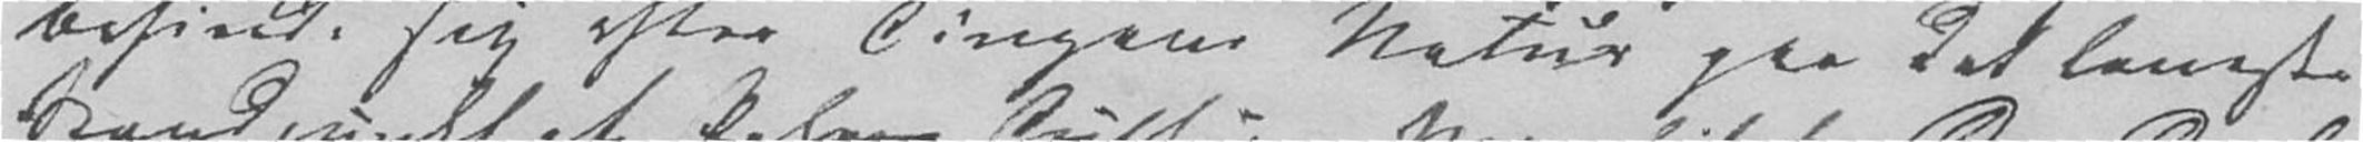befinde sig efter Tingenes Natur paa det laveste
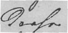Derfor
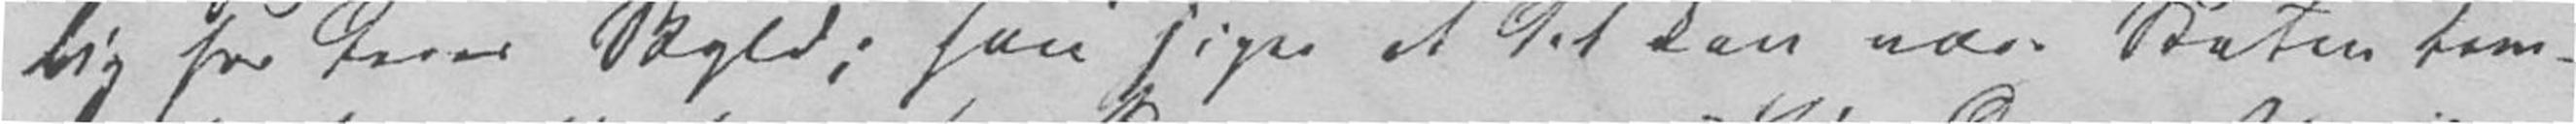lig for deres Skyld; han siger at det kan være Staten tem-
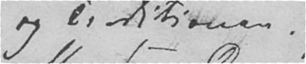og Traditionen.
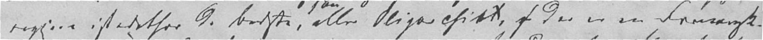regjere istedetfor de bedste, eller Oligarchiet, o der er en Forvansk-
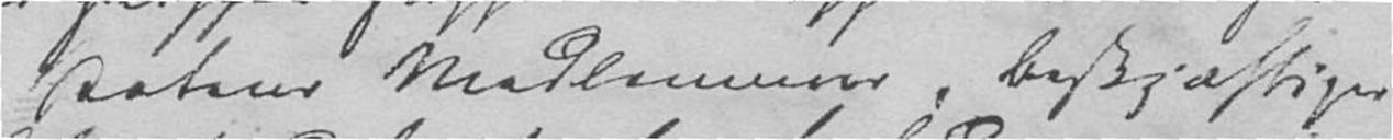Statens Medlemmer, beskjæftiger
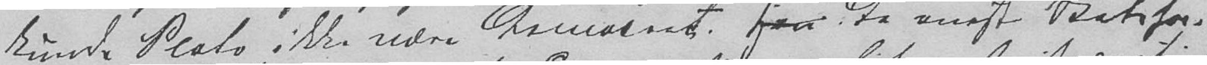kunde Plato ikke være Democrat. Han De eneste Statsfor-
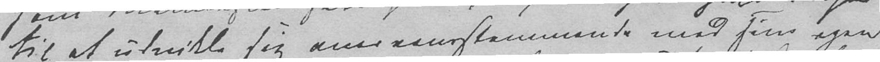til at udvikle sig overeensstemmende med sin egen
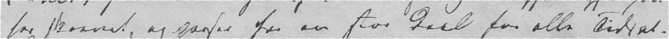har skrevet, og passer for en stor Deel for alle Tidsal-
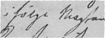ifølge Magten
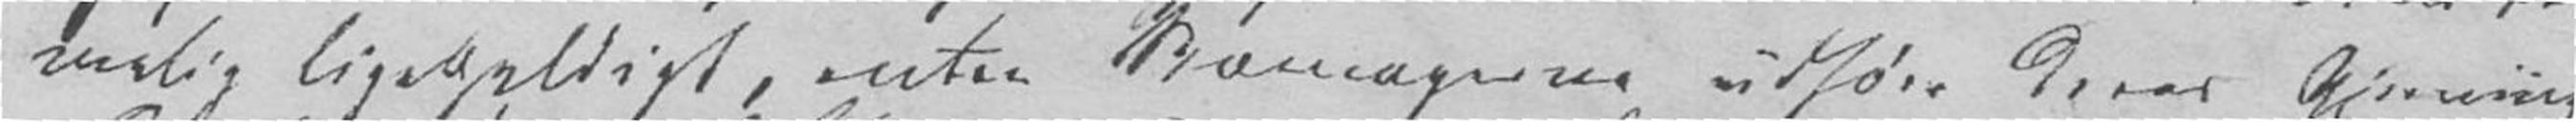melig ligegyldigt, enten Skomagerne udføre deres Gjerning
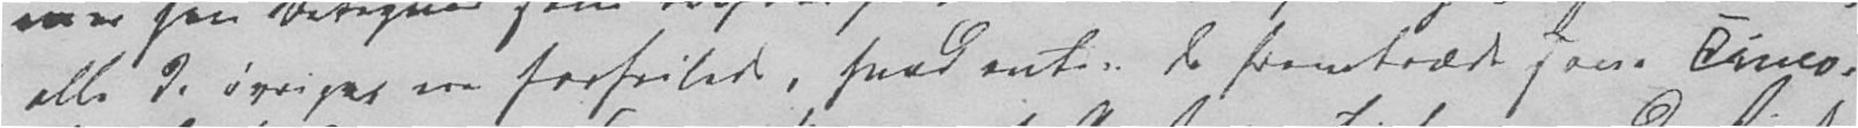alle de øvriges ere forfeilede, hvad enten de fremtræde som Timeo-
